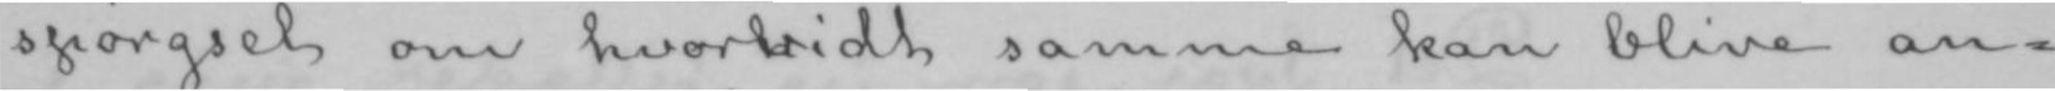spørgsel om hvorvidthvorh samme kan blive an-
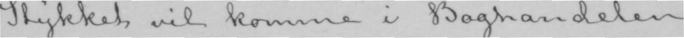Stykket vil komme i Boghandelen
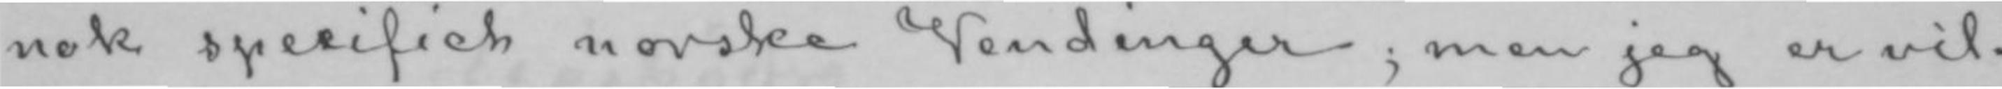nok specifict norske Vendinger; men jeg er vil-
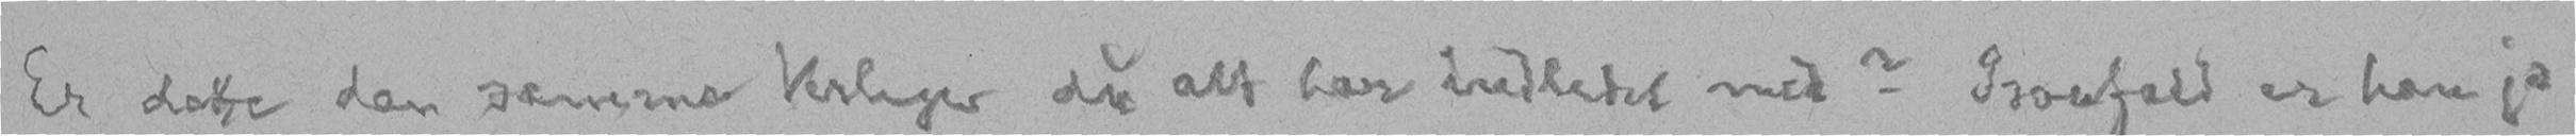Er dette den samme Verleger du alt har indledet med? Isaafald er han jo
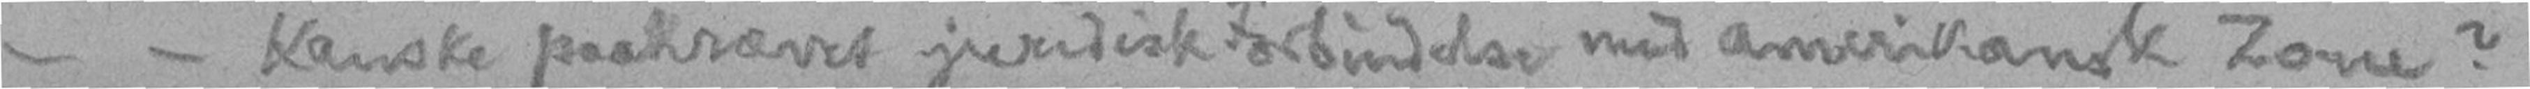- - Kanske paakrævet juridisk Forbindelse med amerikansk Zone?
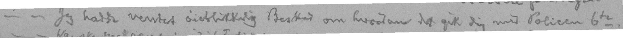- - Jeg hadde ventet øieblikkelig Besked om hvordan det gik dig med Policen 6te.
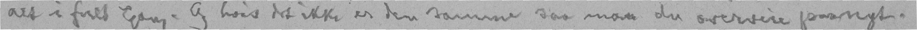alt i fuld Gang. Og hvis det ikke er den samme saa maa du overveie paanyt.
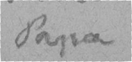Papa
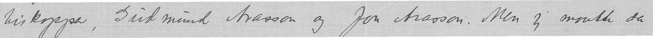biskopper, Gudmund Arasson og Jon Arasson. Men jeg maatte da
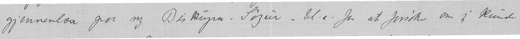gjennemlæse paa ny Biskupa Søgur – bl.a. for at forsøke om jeg kunde
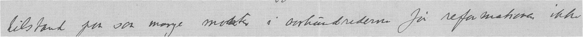tilstand paa saa mange maater i aarhundrederne før reformationen ikke
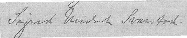Sigrid Undset Svarstad.
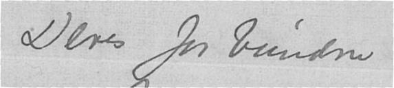Deres forbundne
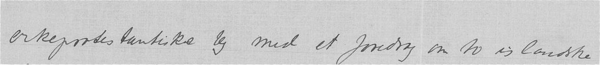erkeprotestantiske by med et foredrag om to islandske
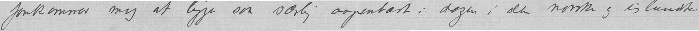forekommer mig at ligge saa særlig aapenbart i dagen i den norske og islandste
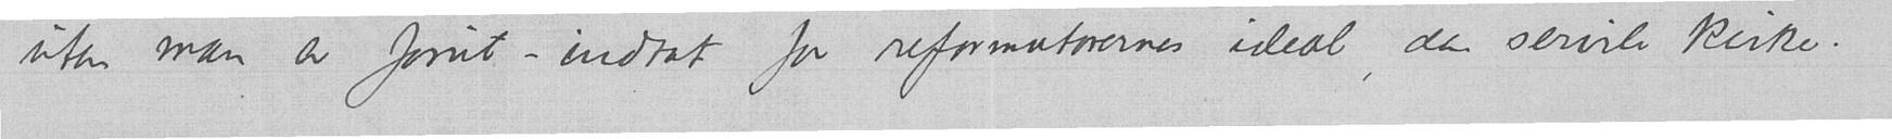uten man er forut-indtat for reformatorernes ideal, den servile kirke.
